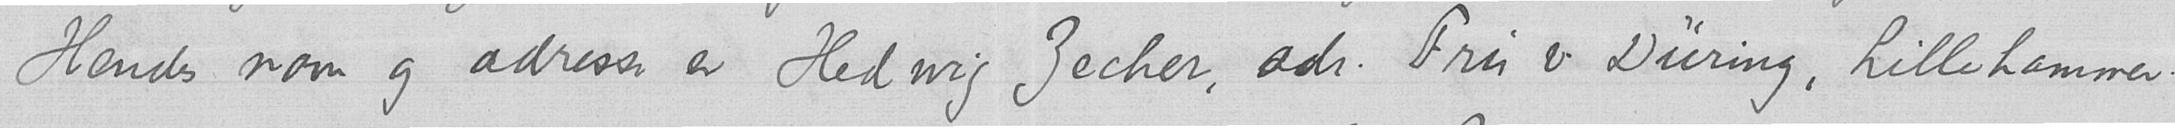Hendes navn og adresse er Hedwig Zecher, adr. Fru v Düring, Lillehammer.
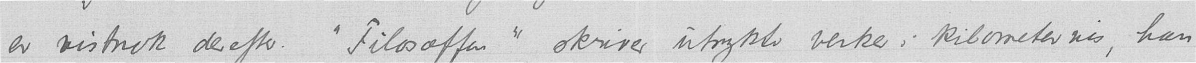er vistnok derefter. "Filosoffen" skriver utrykte verker i kilometervis, han
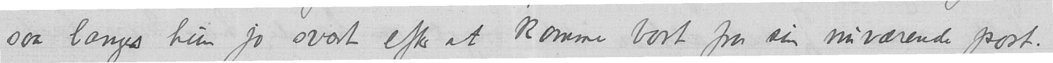saa længes hun jo svært efter at komme bort fra sin nuværende post.
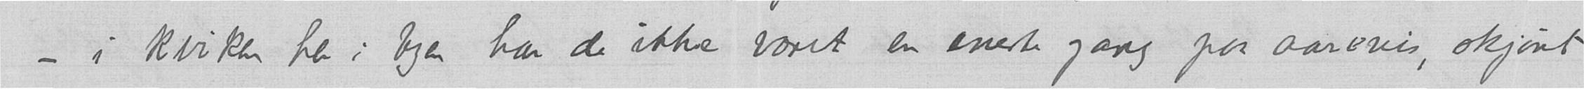– i kirken her i byen har de ikke været en eneste gang paa aarevis, skjønt
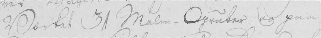Værket 31 Malm-Gruber og paa
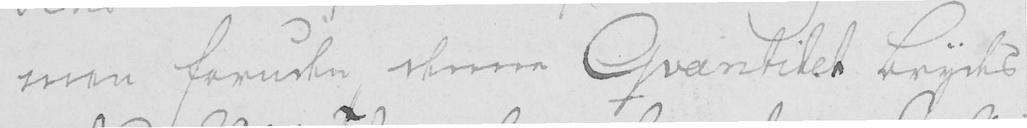men foruden denne Qvantitet brydes
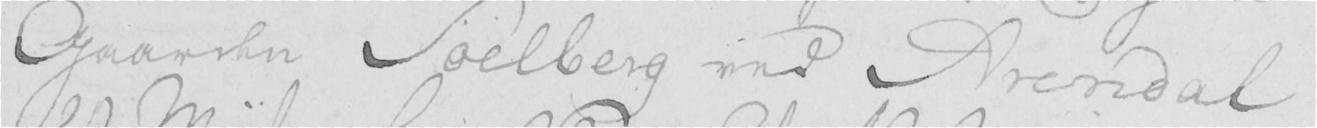Gaarden Soelberg ved Arendal
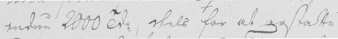endnu 2000 Tdr, deels for at erstatte
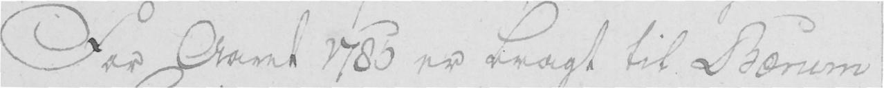For Aaret 1780 er bragt til Bærum
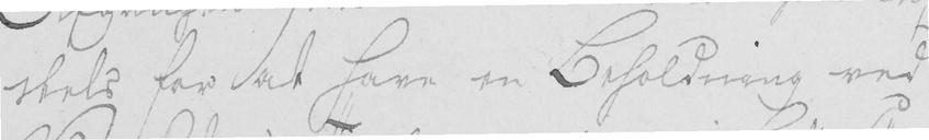deels for at have en Beholdning ved
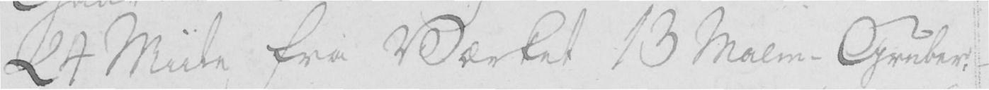24 Miile fra Værket 13 Malm-Gruber -
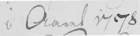i Aaret 1778.
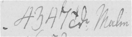4347 Tdr Malm
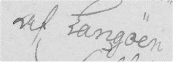af Langøen
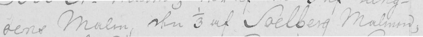øens Malm, den 1/3 af Soelberg Malmen,
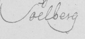Soelberg
